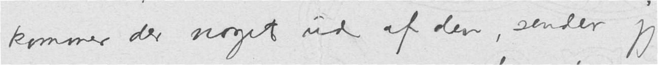kommer der noget ud af den, sender jeg
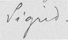Sigrid.
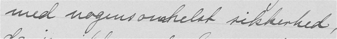med nogensomhelst sikkerhed,
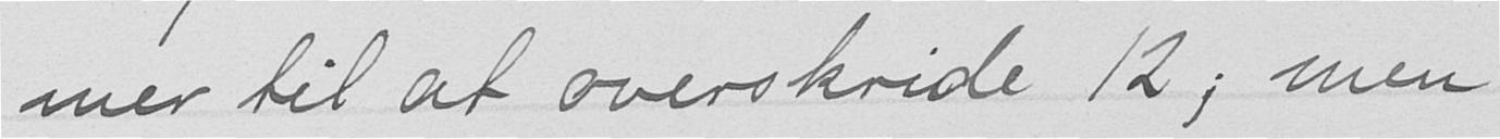mer til at overskride 12; men
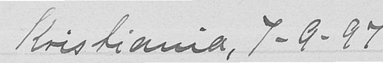Kristiania, 7-9-97
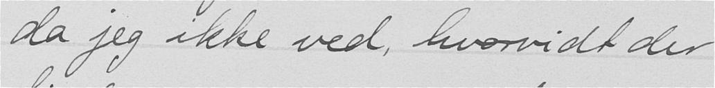da jeg ikke ved, hvorvidt der
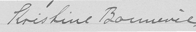Kristine Bonnevie
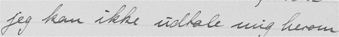jeg kan ikke udtale mig herom
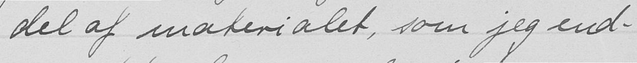del af materialet, som jeg end-
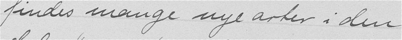findes mange nye arter i den
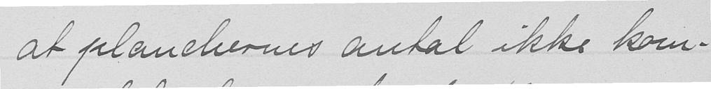at planchernes antal ikke kom-
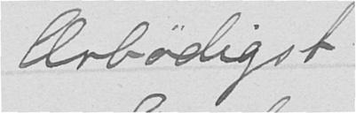Ærbødigst
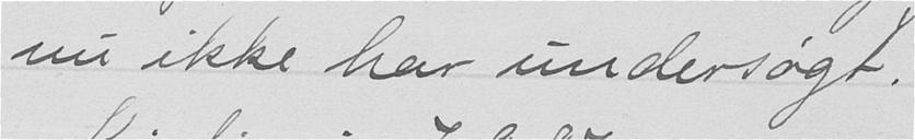nu ikke har undersøgt.
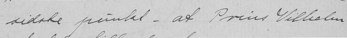sidste punkt - at Prins Vilhelm
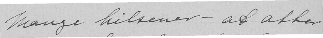Mange hilsener - at atter
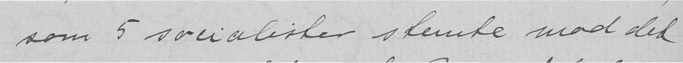som 5 socialister stemte mod det
